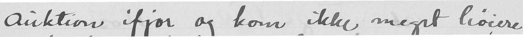auktion ifjor og kom ikke meget høiere
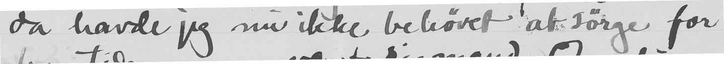da havde jeg nu ikke behøvet at sørge for
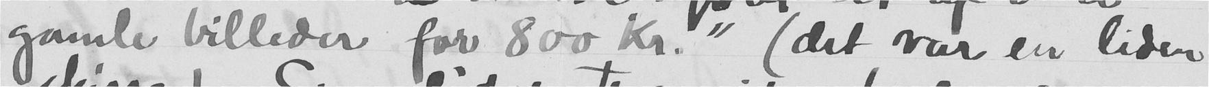gamle billeder for 800 kr." (det var en liden
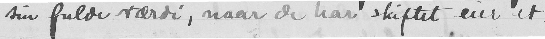sin fulde værdi, naar de har skiftet eier et
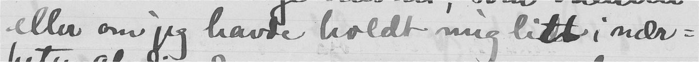eller om jeg havde holdt mig lidt i nær-
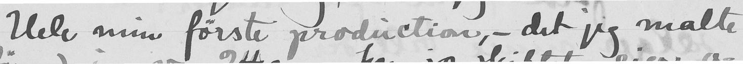Hele min første production, - det jeg malte
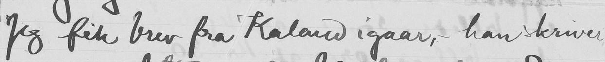Jeg fik brev fra Kaland igaar, - han skriver
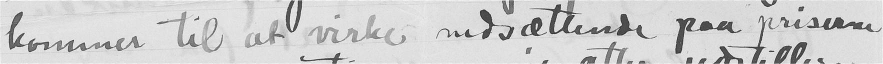kommer til at virke nedsættende paa priserne
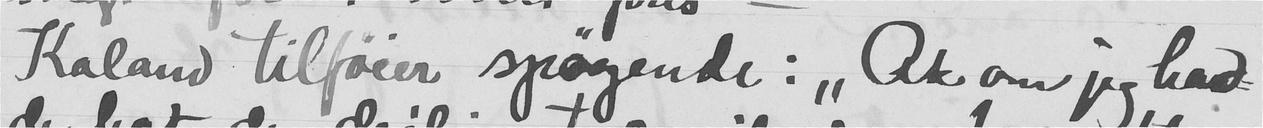Kaland tilføier spøgende: "Ak om jeg had-
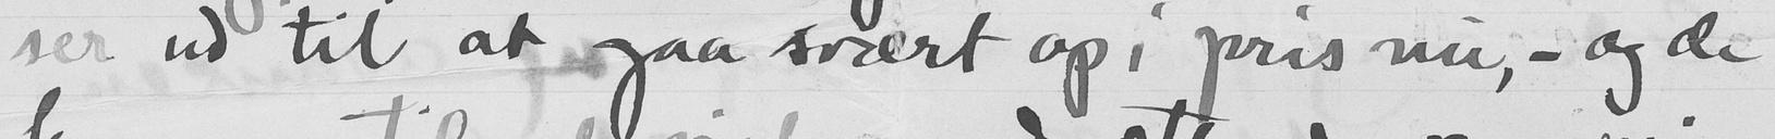ser ud til at gaa svært op i pris nu, - og de
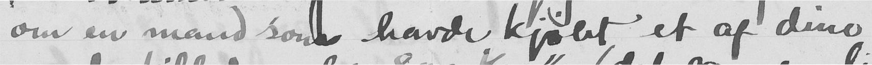om en mand som havde kjøbt et af dine
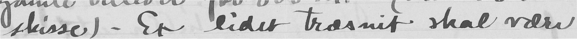skisse) - Et lidet træsnit skal være
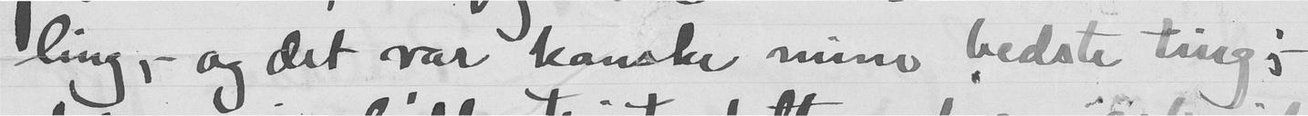ling,- og det var kanske mine bedste ting; -
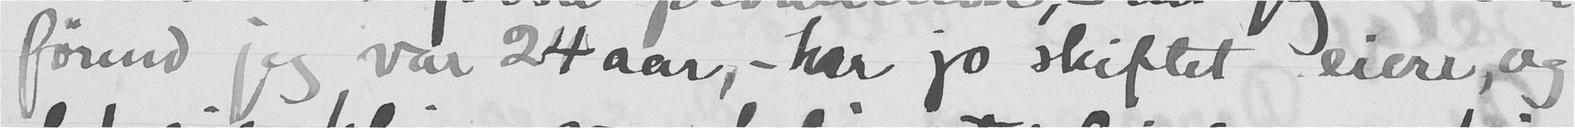førend jeg var 24 aar, - har jo skiftet eiere, og
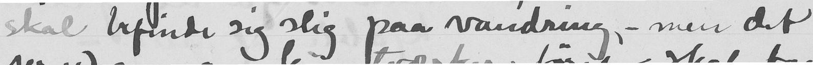skal befinde sig slig paa vandring, - men det
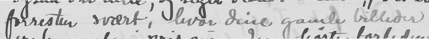forresten svært, hvor dine gamle billeder
